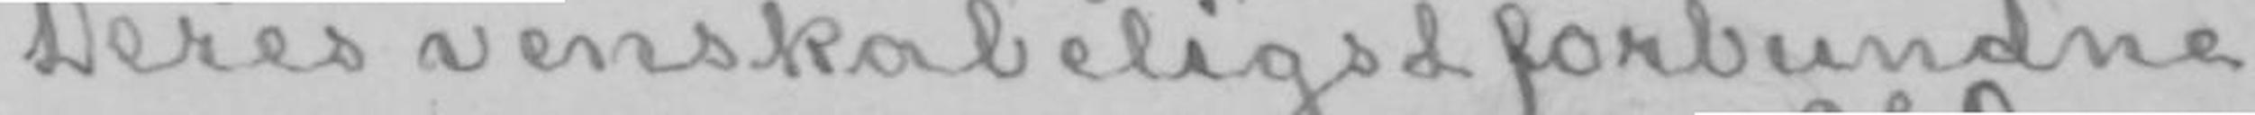Deres venskabeligst forbundne
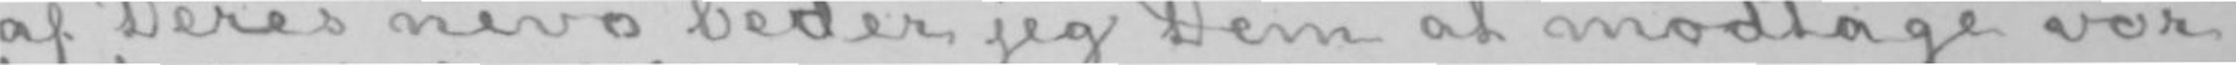af Deres nevø beder jeg Dem at modtage vor
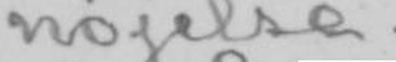nøjelse.
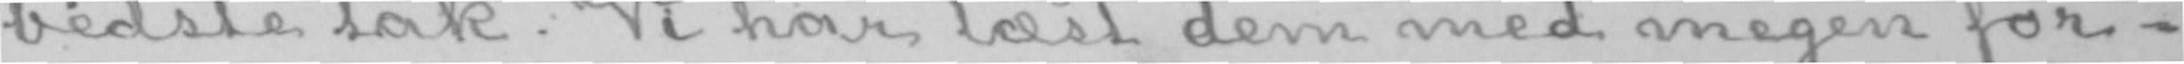bedste tak. Vi har læst dem med megen for-
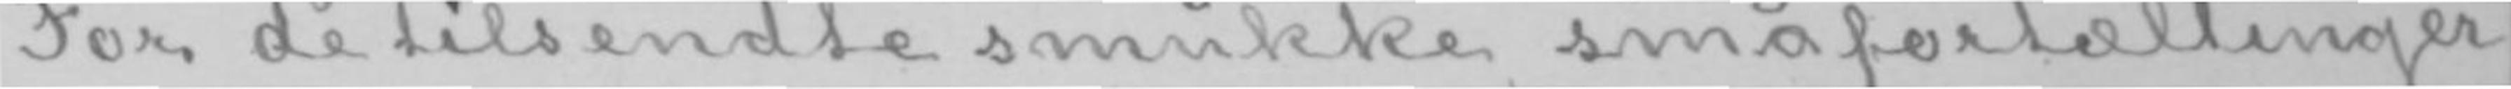For de tilsendte smukke småfortællinger
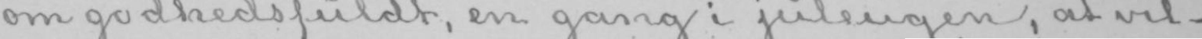om godhedsfuldt, en gang i juleugen, at vil-
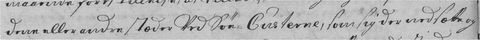dene eller andre stæder ved Søe-Custerne, som sig der nedsætte og
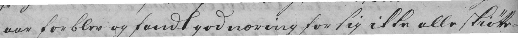aar forblev og fandt god næring for sig ikke alle skiøtte-
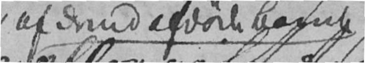af dend afdøde Hamk
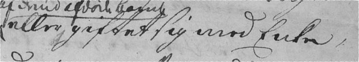eller giftet sig med Enke,
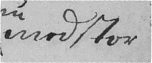med stor
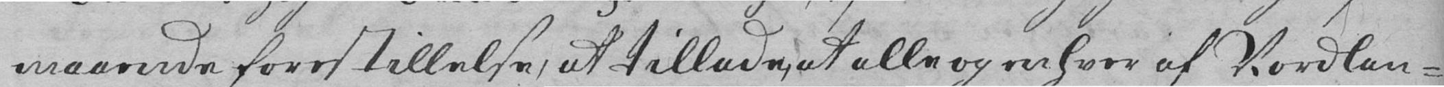maaende forestillelse, at tillade, at alle og enhver af Nordlan-
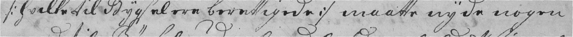/: hvilke til Bygsel ere berettigede :/ maatte nyde nogen
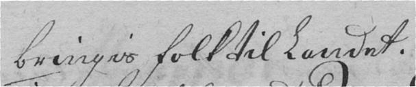bringis Folk til Landet.
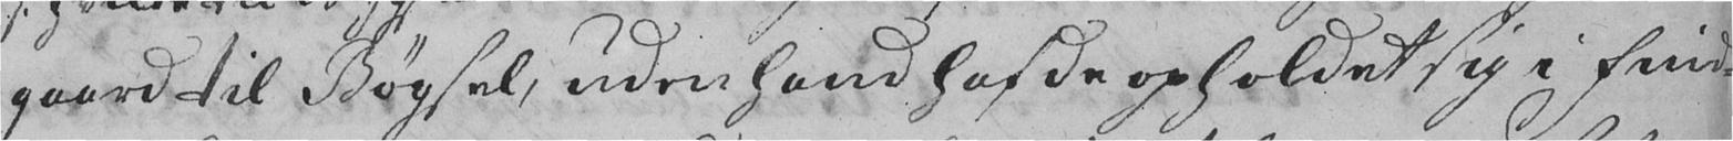gaard til Bøgsel, uden hand hafde opholdet sig i Find-
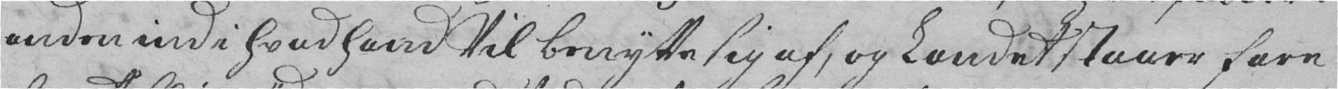anden ind i hvad hand Vil benytte sig af, og Landet staaer fare
